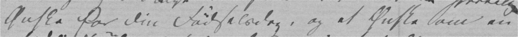Ønske for Din Fødselsdag, og et Ønske om en
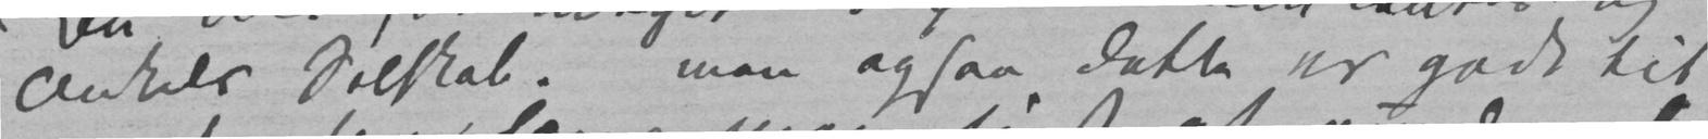Onkels Selskab. men ogsaa dette er godt til
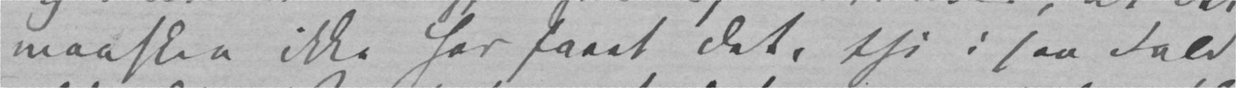maaskee ikke har faaet det, thi i saa Fald
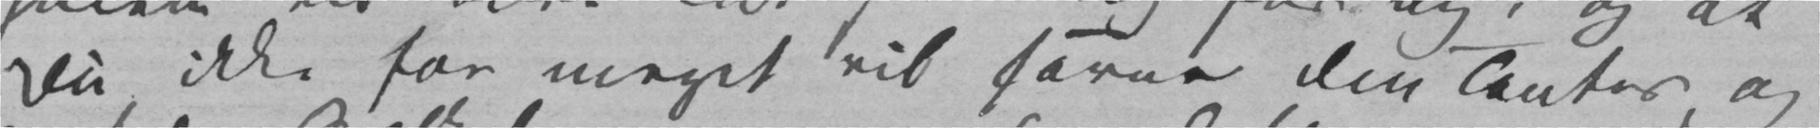Du ikke for meget vil savne Din Tantes og
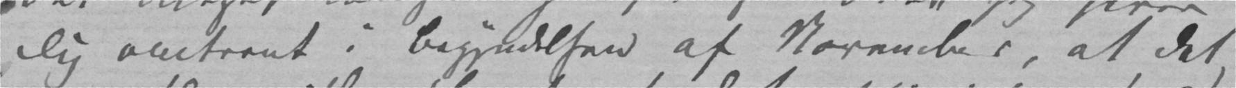Dig omtrent i Begyndelsen af November, at det
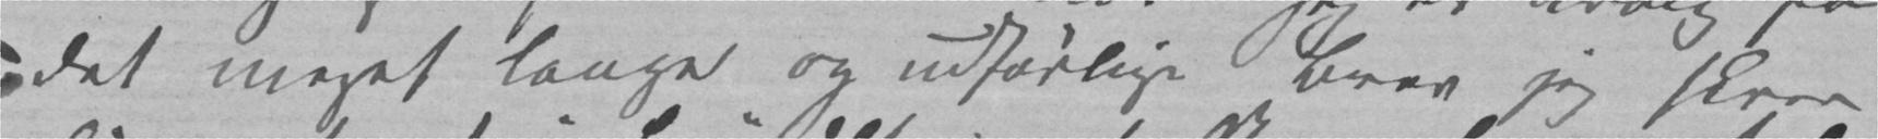det meget lange og udførlige Brev jeg skrev
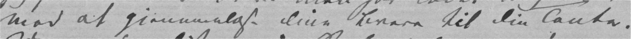med at gjennemlæse Dine Breve til Din Tante.
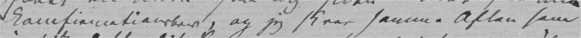Comfirmationsbrev, og jeg skrev samme Aften som
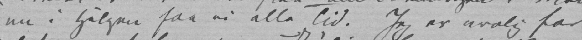nu i Helgen faa vi alle Tid. Jeg er urolig for
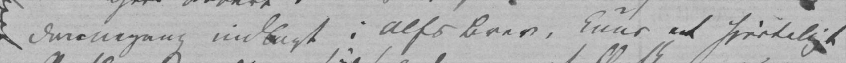dennegang indlagt i Alfs Brev, kuns et hjerteligt
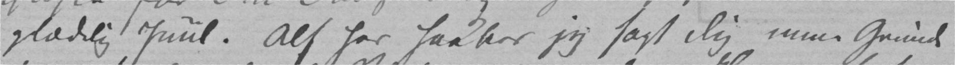glædelig Juul. Alf har haaber jeg sagt Dig mine Grunde
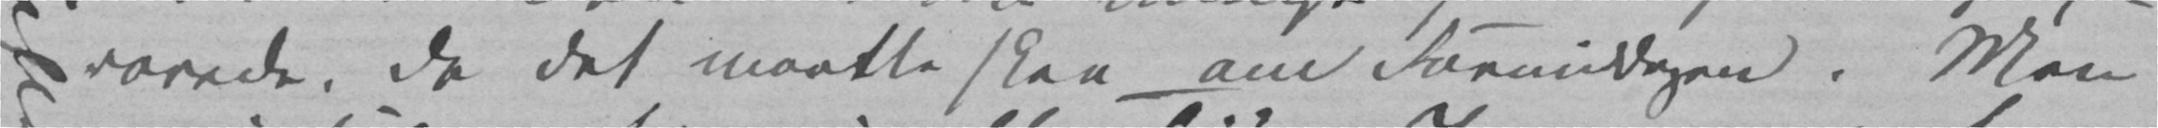varede, da det maatte skee om Formiddagen. Men
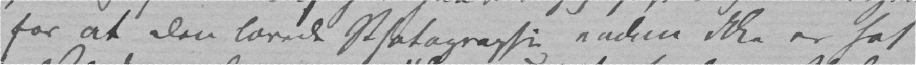for at den lovede Photographie endnu ikke er sat
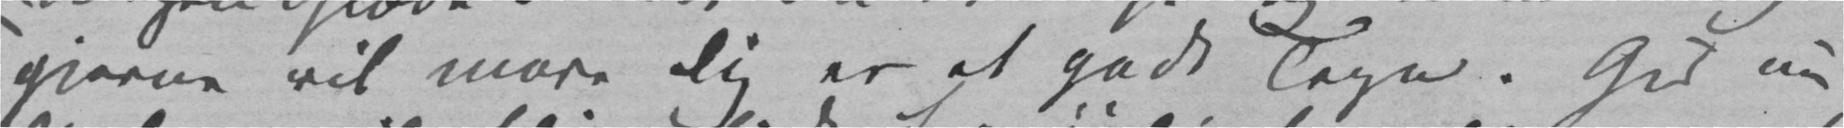gjerne vil more Dig er et godt Tegn. Gid nu
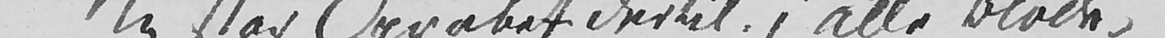Nu står Opråbet dertil i alle Blade,
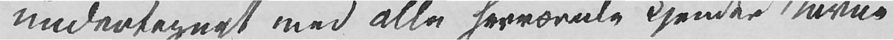undertegnet med alle herværende kjendte Navne
